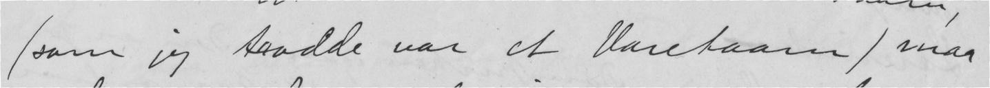(som jeg trodde var et Varetaarn) maa
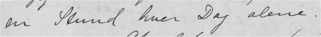en Stund hver Dag alene.
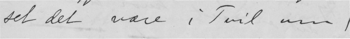set det være i Tvil om
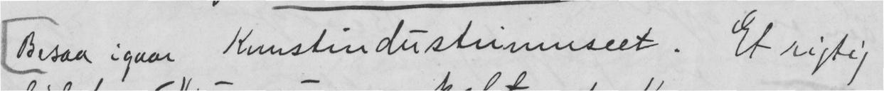Besaa igaar Kunstindustrimuseet. Et rigtig
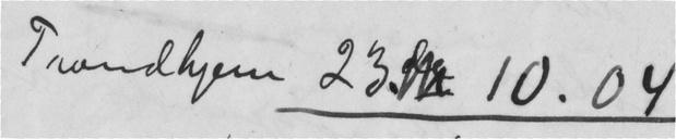Trondhjem 23.  10.04
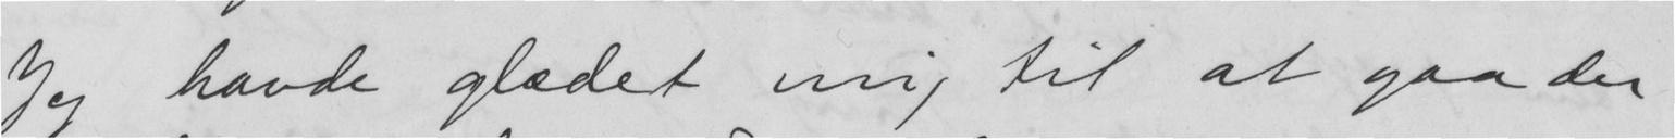Jeg havde glædet mig til at gaa der
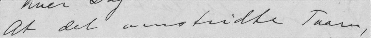At det omstridte Taarn,
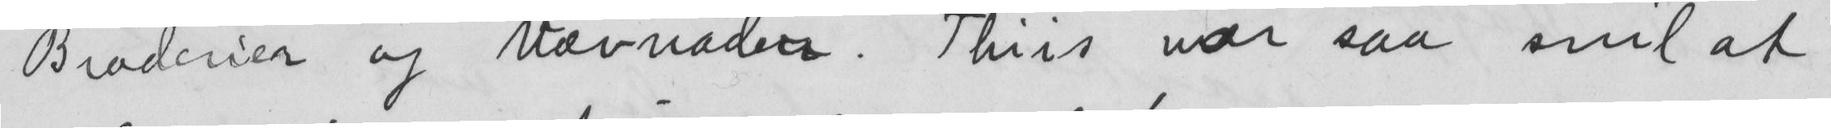Broderier og Vævnader. Thiis var saa snil at
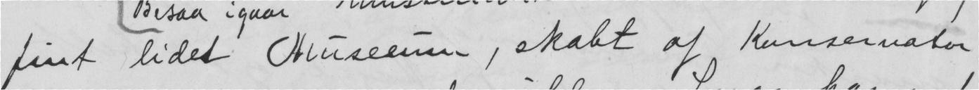fint lidet Museeum, skabt af Konservator
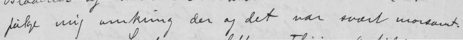følge mig omkring der og det var svært morsomt.
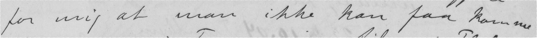for mig at man ikke kan faa komme
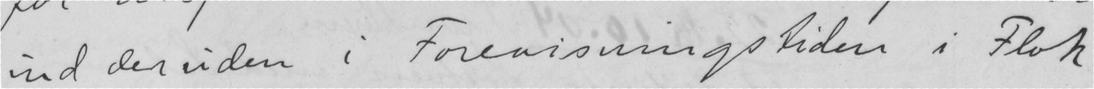ind der uden i Forevisningstiden i Flok
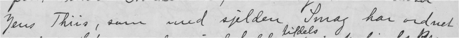Jens Thiis, som med sjelden Smag har ordnet
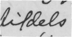tildels
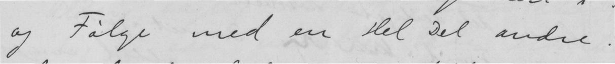og Følge med en Hel Del andre.
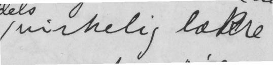virkelig lækre
